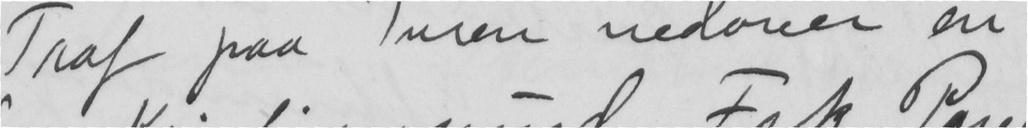Traf paa Turen nedover en
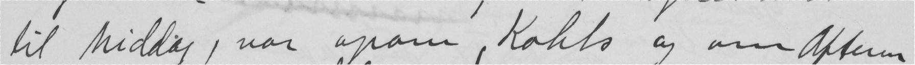til Middag, var opom Kohts og om Aftenen
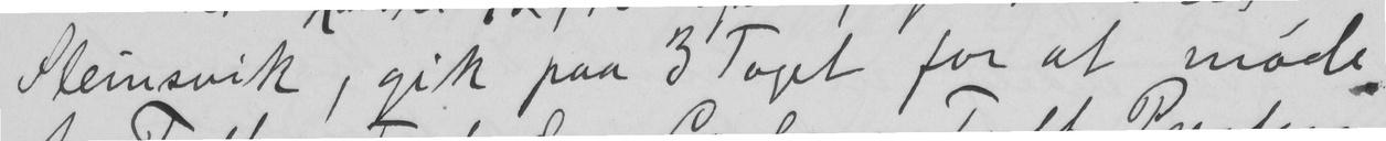Steinsvik, gik paa 3 Toget for at møde
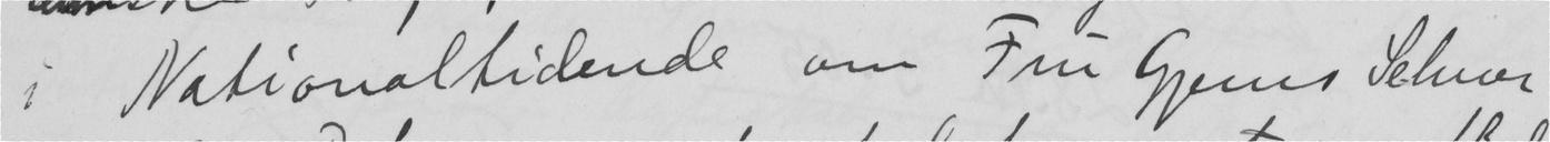i Nationaltidende om Fru Gjems Selmer
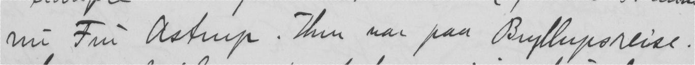nu Fru Astrup. Hun var paa Bryllupsreise.
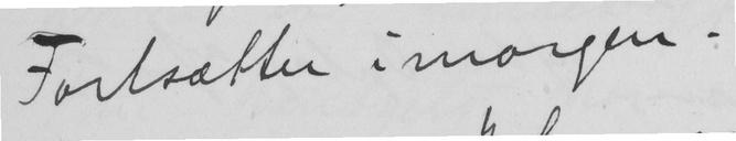Fortsætter imorgen.
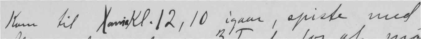Kun til Xania kl. 12, 10 igaar, spiste med
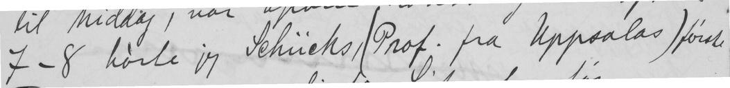7-8 hørte jeg Schücks (Prof. fra Uppsalas) første
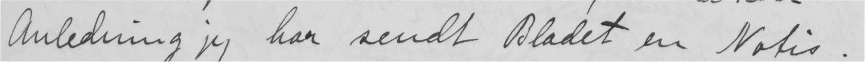Anledning jeg har sendt Bladet en Notis.
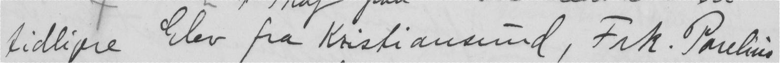tidligere Elev fra Kristiansund, Frk. Parelius
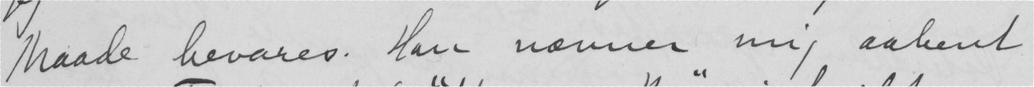Maade bevares. Han nævner mig aabent
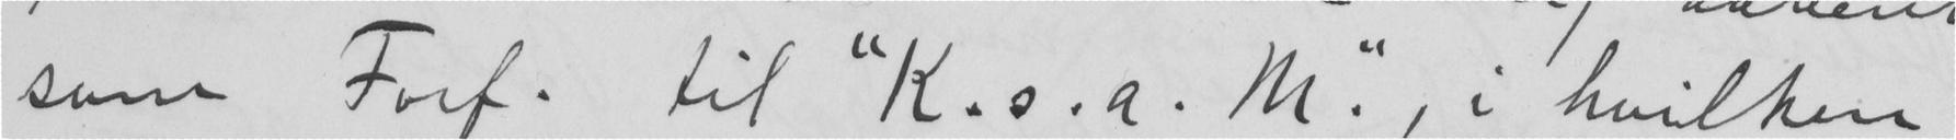som Forf. til "K. s. a. M.", i hvilken
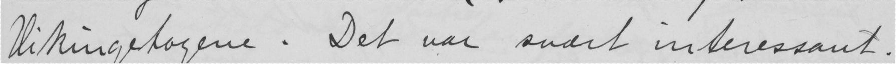Vikingetogene. Det var svært interessant.
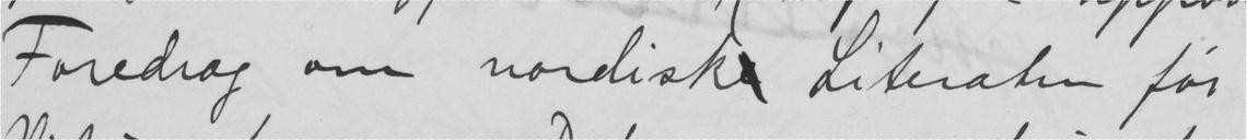Foredrag om nordisk Literatur før
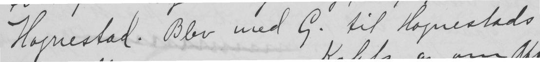Hognestad. Blev med G. til Hognestads
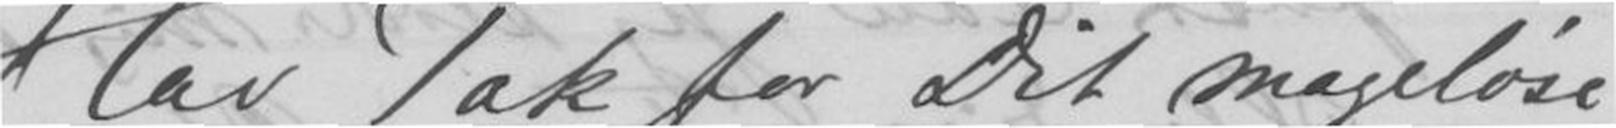Hav Tak for Dit mageløse
